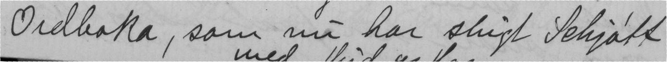Ordboka, som nu har slugt Schjøtt
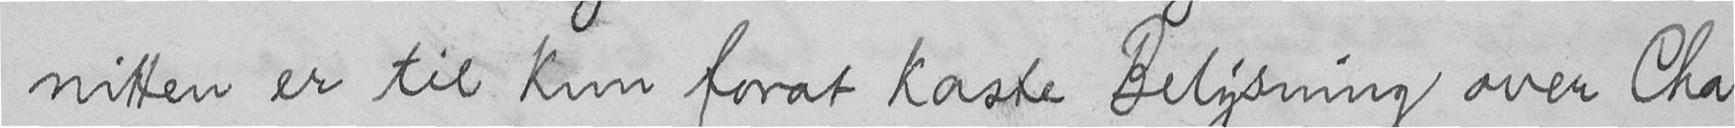nitten er til kun forat kaste Belysning over Cha
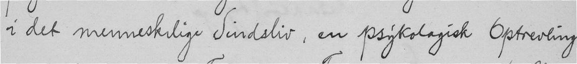i det menneskelige Sindsliv, en psykologisk Optrevling
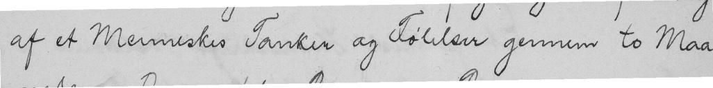af et Menneskes Tanker og Følelser gennem to Maa
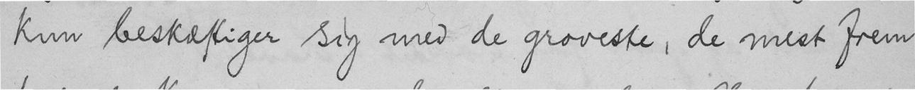kun beskæftiger sig med de groveste, de mest frem
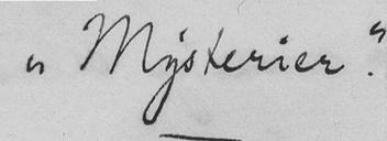"Mysterier".
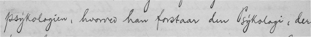psykologien, hvorved han forstaar den Psykologi, der
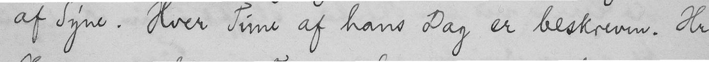af Syne. Hver Time af hans Dag er beskreven. Hr
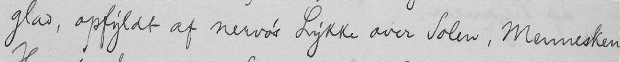glad, opfyldt af nervøs Lykke over Solen, Mennesken
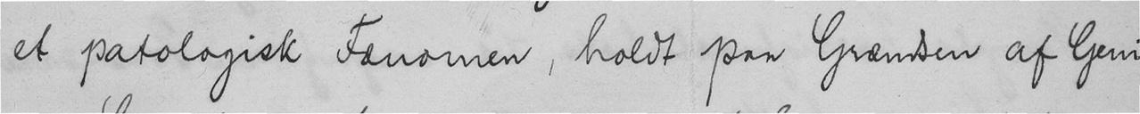et patologisk Fænomen, holdt paa Grændsen af Geni
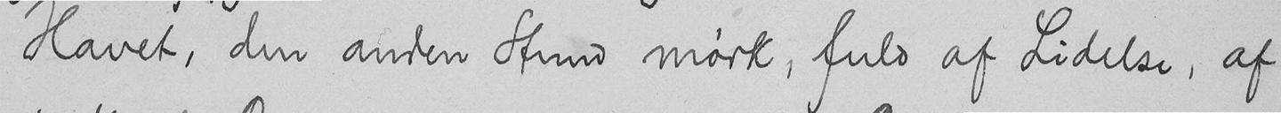Havet, den anden Stund mørk, fuld af Lidelse, af
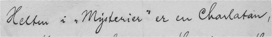Helten i "Mysterier" er en Charlatan,
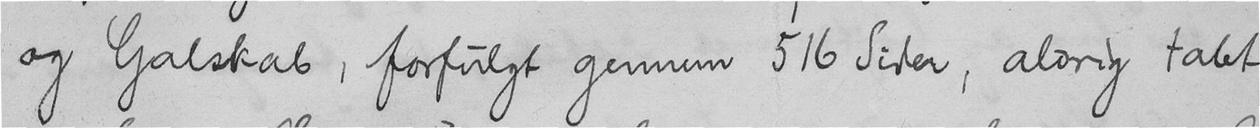og Galskab, forfulgt gennem 516 Sider, aldrig tabt
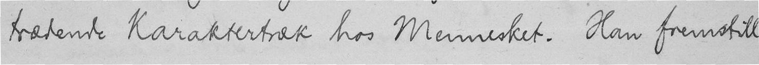trædende Karaktertræk hos Mennesket. Han fremstill
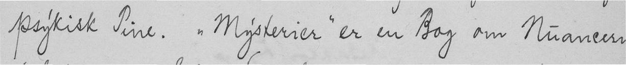psykisk Pine. "Mysterier" er en Bog om Nuancern
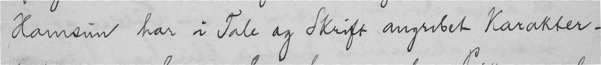Hamsun har i Tale og Skrift angrebet Karakter-
